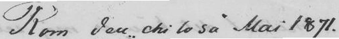Rom, den "chi lo sa" Mai 1871.
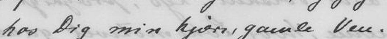hos Dig min kjære, gamle Ven.
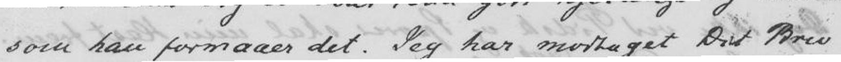som han formaar det. Jeg har modtaget Dit Brev
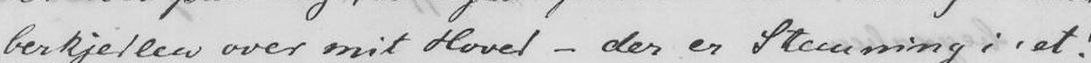berkjedlen over mit Hoved - der er Stemning i et!
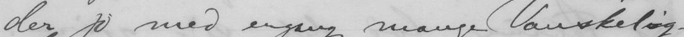der jo med engang mange Vanskelig-
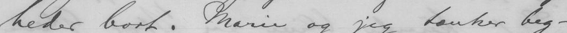heder bort. Marie og jeg tænker beg-
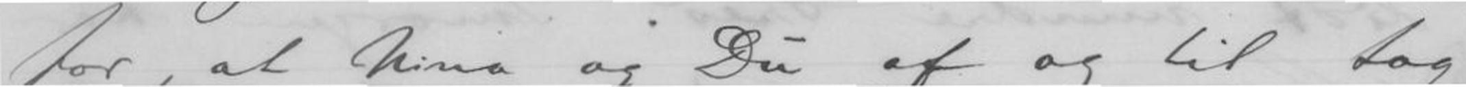for, at Nina og Du af og til tog
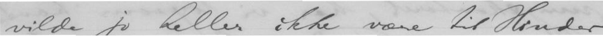vilde jo heller være til Hinder
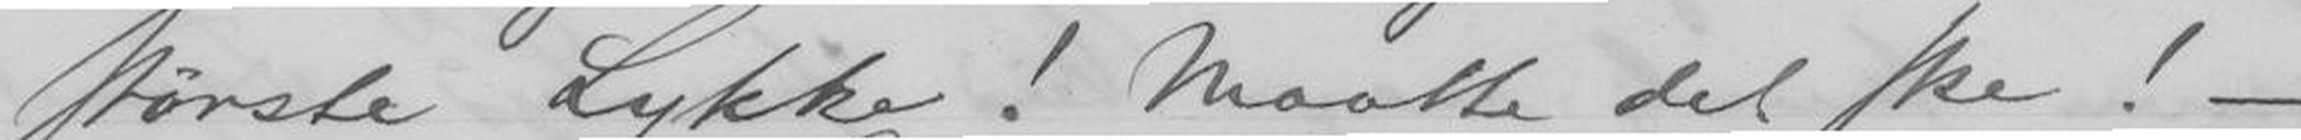største Lykke! Maatte det ske! -
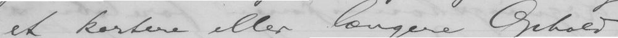et kortere eller længere Ophold
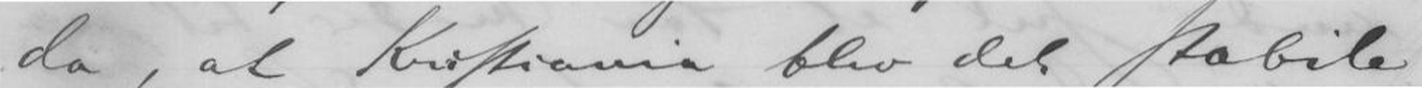da, at Kristiania blev det stabile
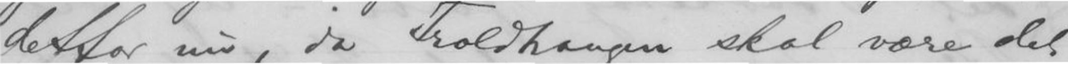detfor nu, da Troldhaugen skal være det
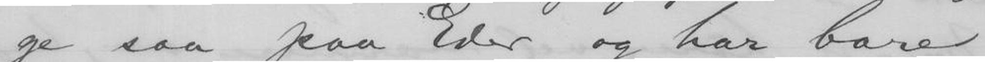ge saa paa Eder og har bare
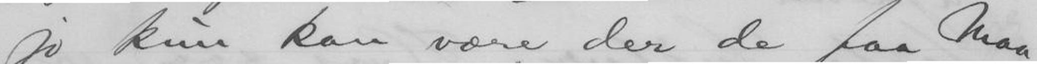jo kun kan være der de faa Maa-
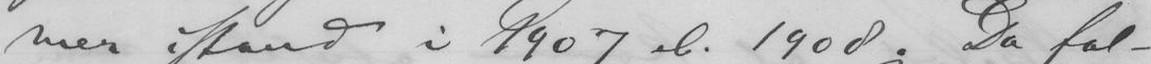mer istand i 1907 el. 1908. Da fal-
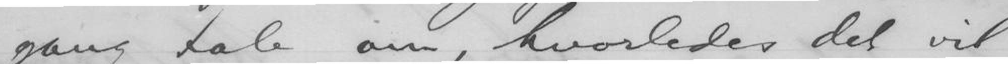gang tale om, hvorledes det vil
